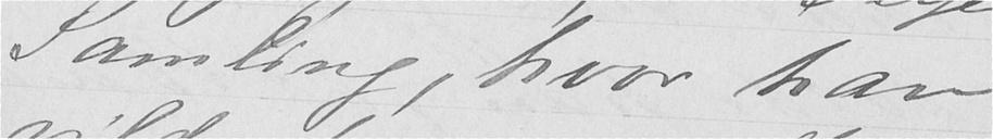Samling, hvor han
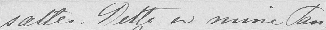sættes. Dette er mine Tan-
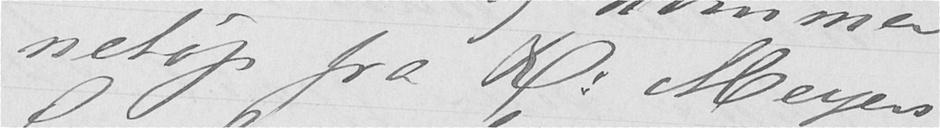netop fra R. Meyers
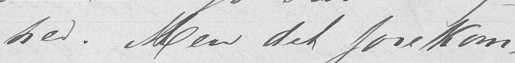hed. Men det forekom-
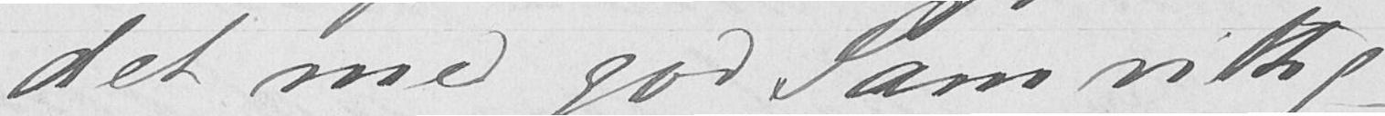det med god samvittig-
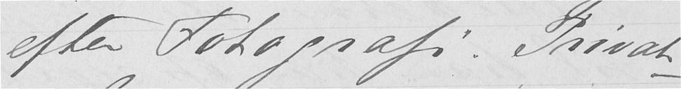efter Fotografi. Privat-
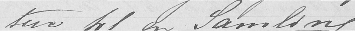tur til en Samling
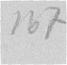187.
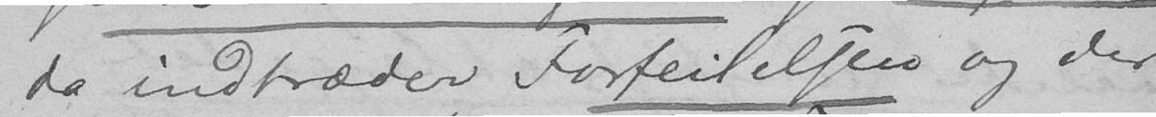da indtræder Forfeilelsen og der
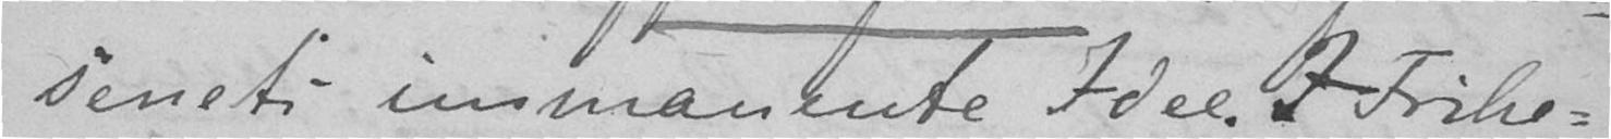senet immanente Idee. I Frihe-
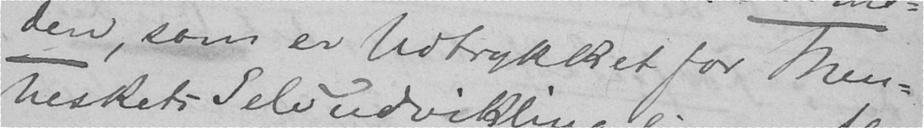den, som er Udtrykket for Men-
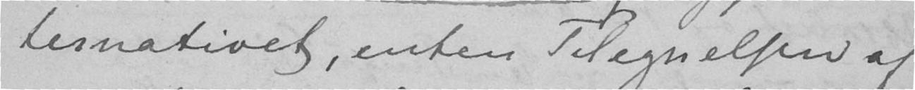ternativet, enten Tilegnelsen af
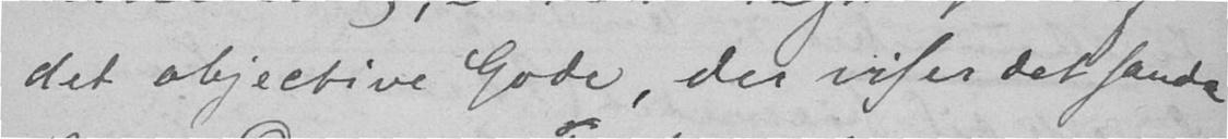det objective Gode, der viser det sande
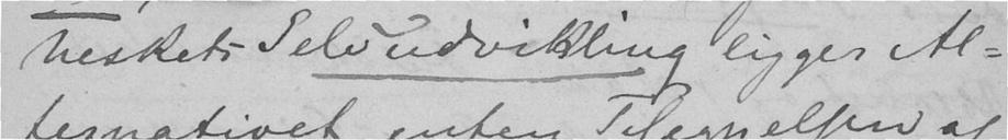neskets Selvudvikling ligger Al-
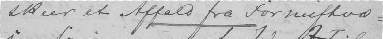skeer et Affald fra Fornuftvæ-
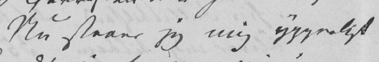Nu staaer jeg mig ypperligt
